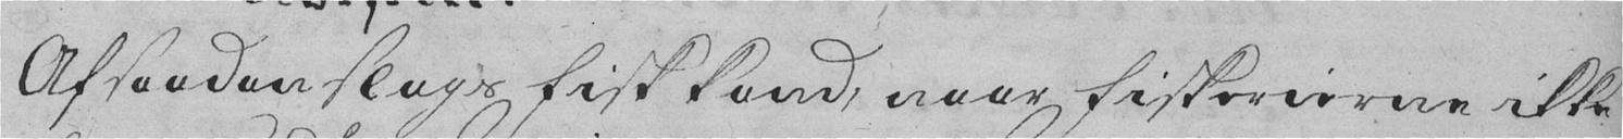Af saadan slags fisk kand, naar Fiskerierne ikke
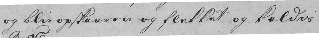og blir opskaaren og flekket og kaldis
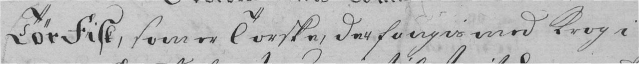Tørfisk, som er Torske, der fangis med Krog i
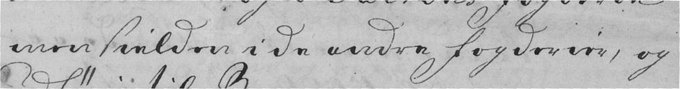men sielden i de andre Fogderier, og
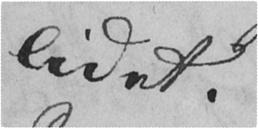lidet.
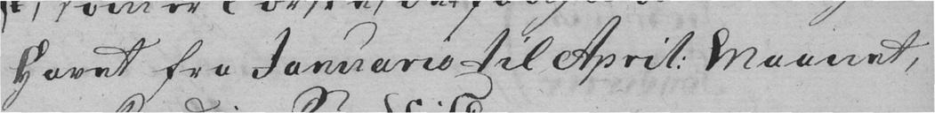Havet fra Ianuario til April Maanet,
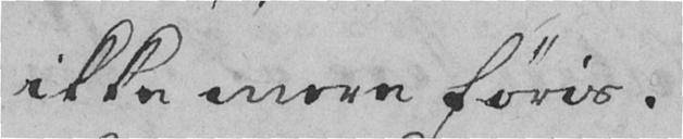ikke mere føris.
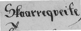Skaarreqveite
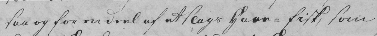saa og for en deel af et slags Haae-fisk, som
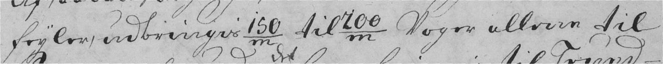feyler, udbringis 150 m til 200 m Voger allran til
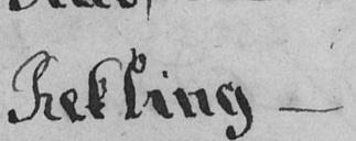Rekling -
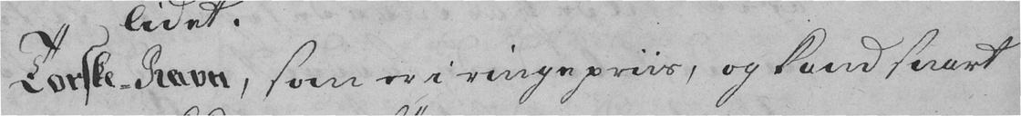Torske-Ravn, som er i ringe priis, og kand snart
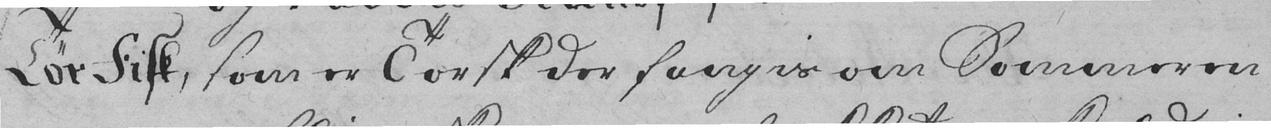Tørfisk, som er Torsk der fangis om Sommeren
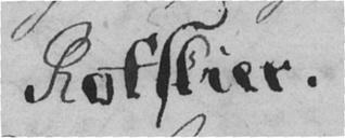Rotskier.
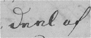deel af.
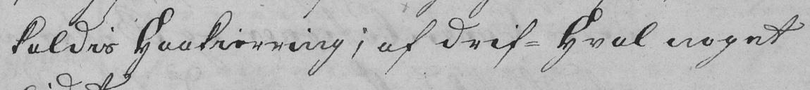kaldes Haakierring; af drif-Hval noget
